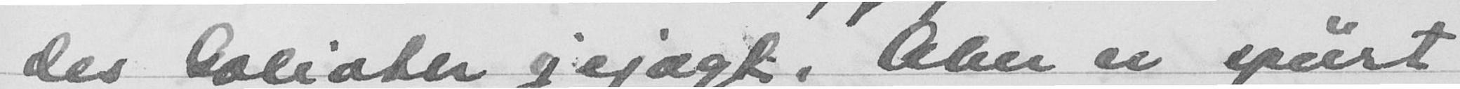des soliater gejagt, Abler er spurt
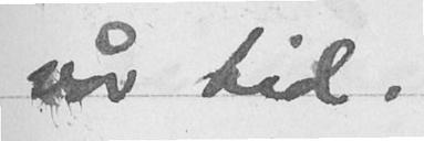vår tid.
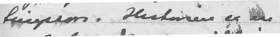tepton. Historien er en
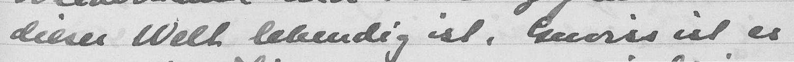dieser Welt lebendig øst. Guviss iit ei
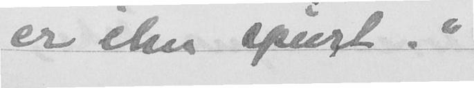er eln spurt.?
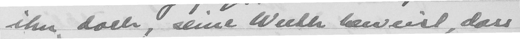ilm doel, seine Wieth hencist, "dæss
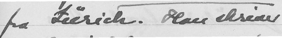fra Zürich. Han skriver
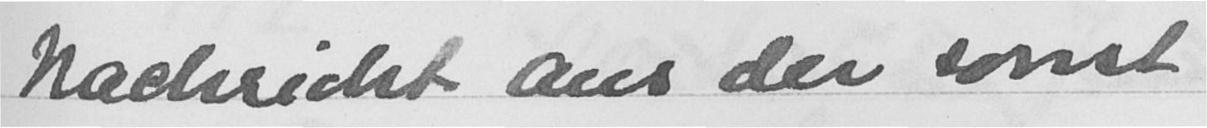Naelsricht ans der somst
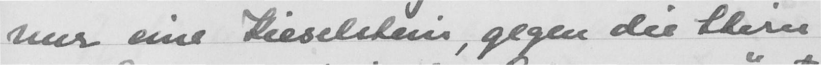mur eine Rieselstern, gigen du Stern
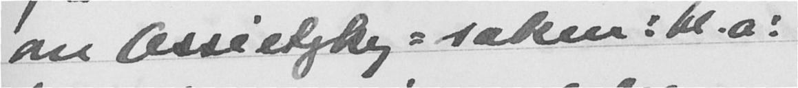om Ossietzky-saken: bl. a:
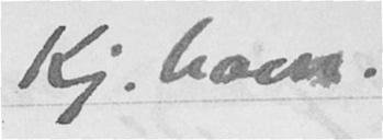Kj. havn.
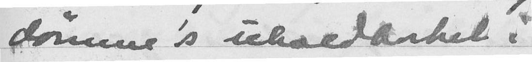dømme's uholdbarhet i
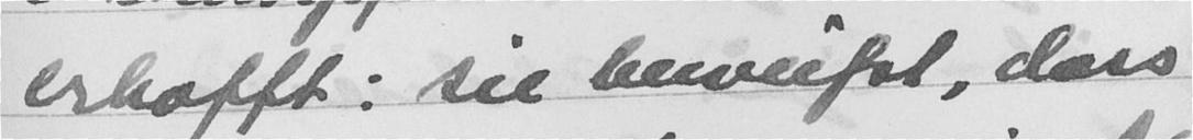erhafft; sie beveifst, dass
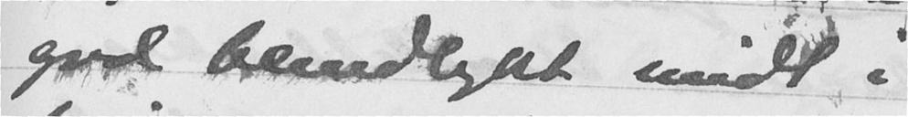gud bæaandliykt midt i
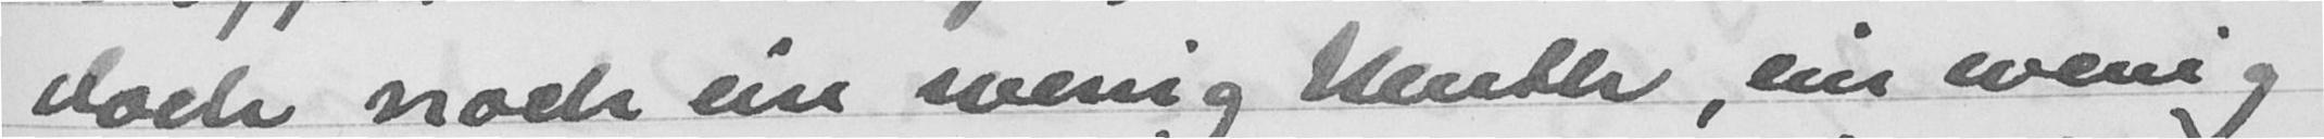doen nioch ein sveriig lenter, enr eveng
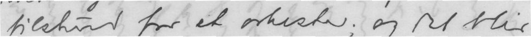tilskud for et orkester; og det blir
